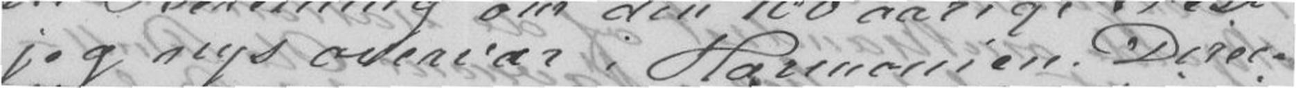jeg nys overvar i Harmonien. Direc-
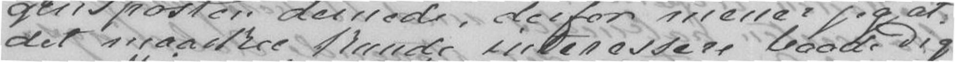det maaskee kunde interessere baade Dig
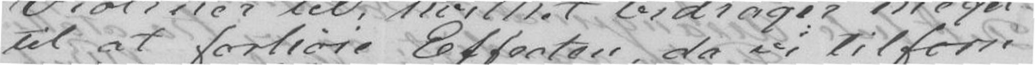til at forhøie Effecten, da vi tilforn
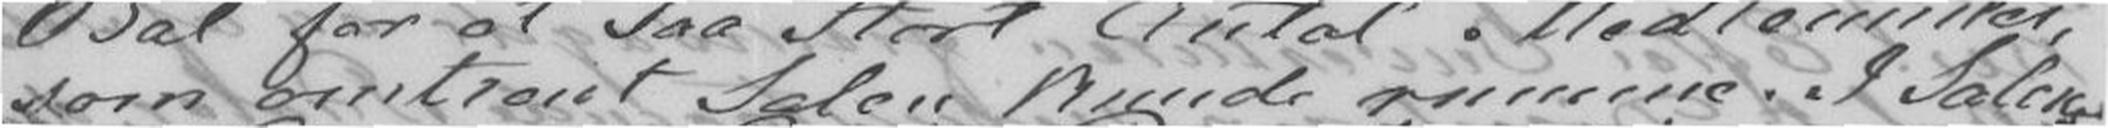som omtrent Salen kunde rumme. I Salen
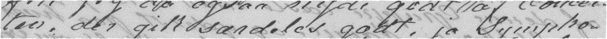ten, der gik særdeles godt, jo Sympho-
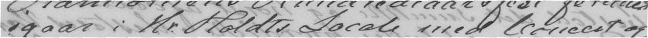igaar i Hr. Holdts Locale med Concert og
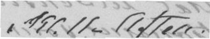Kl.11. Aften.
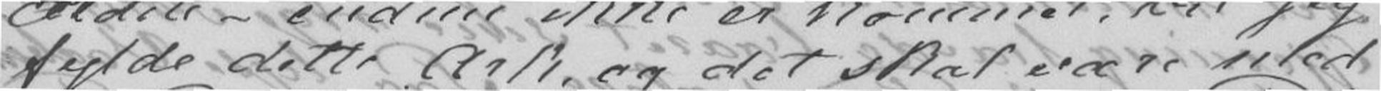fylde dette Ark, og det skal være med
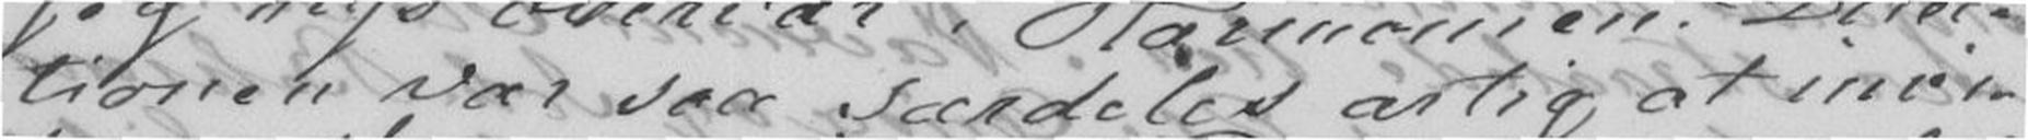tionen var saa særdeles artig at invi-
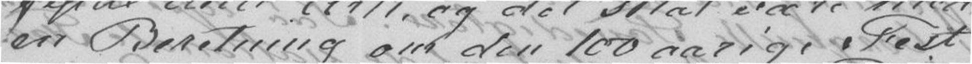en Beretning om den 100 aarige Fest
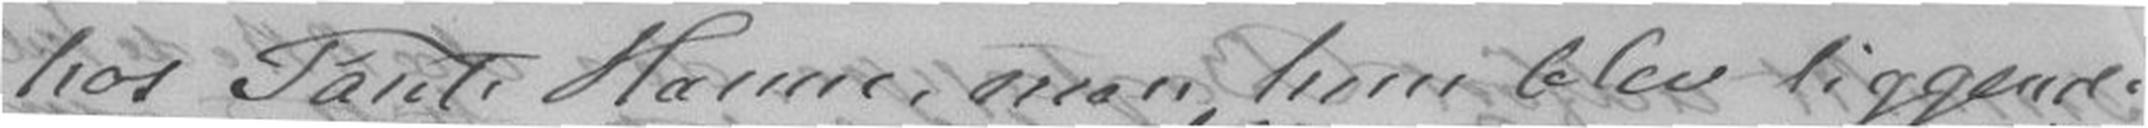hos Tante Hanne, men hun blev ligende
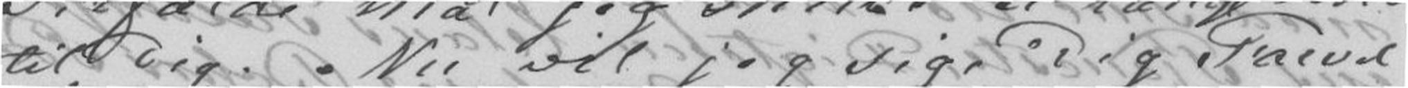til Dig. Nu vil jeg sige Dig Farvel
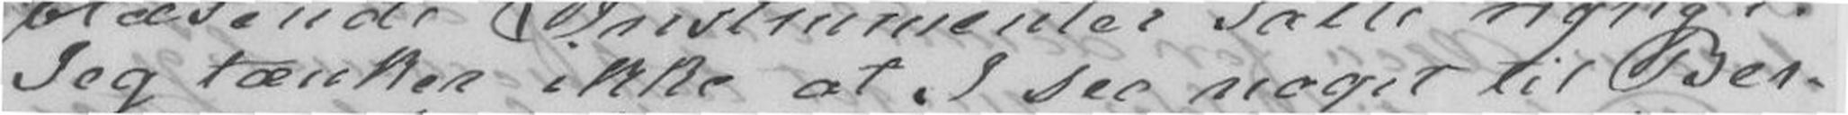Jeg tænker ikke at I saa noget til Ber-
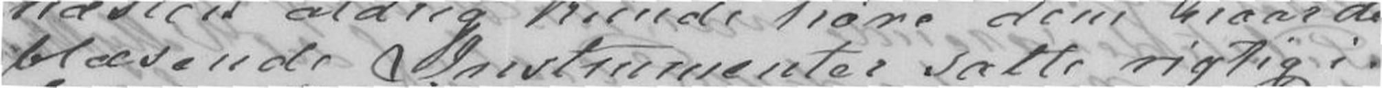blæsende Instrumenter satte rigtig i.
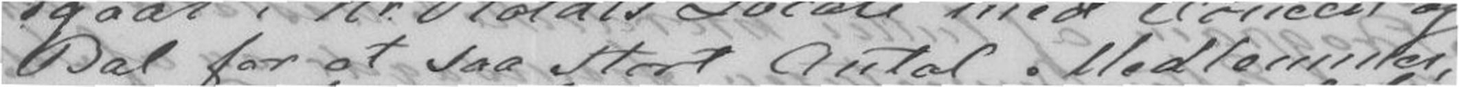Bal for at saa stort Antal Medlemmer,
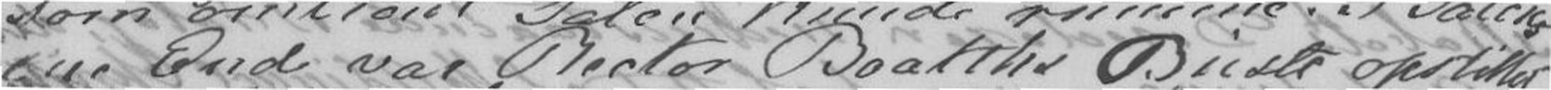ene End var Rector Boalthe Büste opstillet
